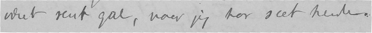været rent gal, naar jeg har sæt hende.
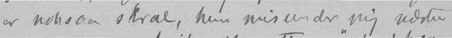er noksaa skral, hun misunder mig næsten
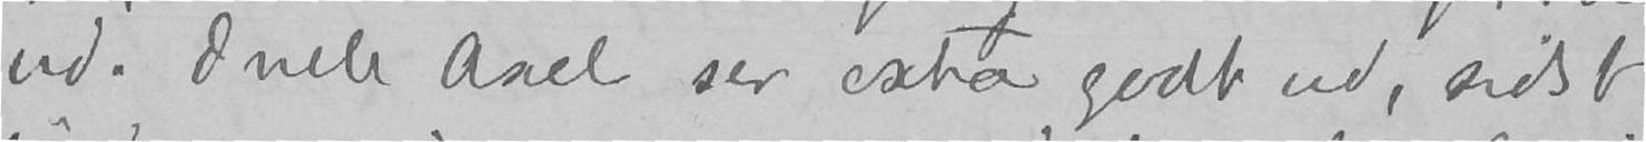ud. Oncle Axel ser extra godt ud, sidst
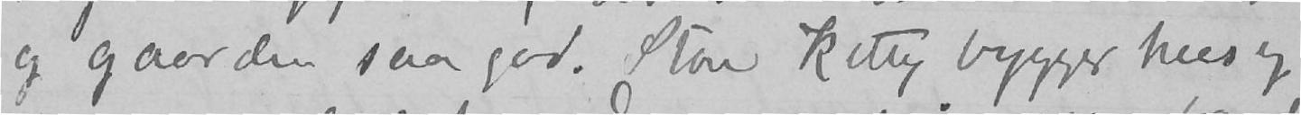og gaarden saa god. Store Kitty bygger hus og
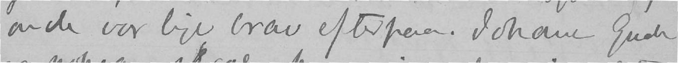oncle var lige brav efterpaa. Johane Gude
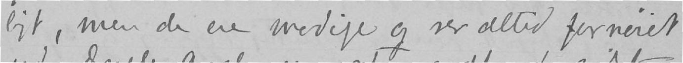ligt, men de ere modige og ser altid fornøiet
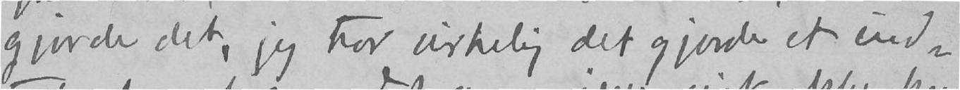gjorde det, jeg tror virkelig det gjorde et ind-
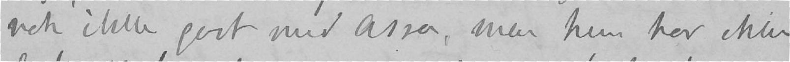nok ikke godt med Assa, men hun har ikke
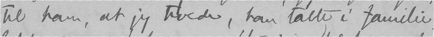til ham, at jeg troede, han tabte i familie-
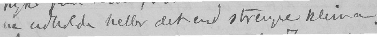ne udholde heller det end strengere klima.
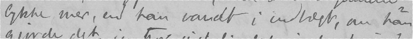lykke mer, end han vandt i indtægt, om han
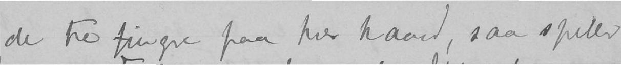de tre fingre paa hver haand, saa spiller
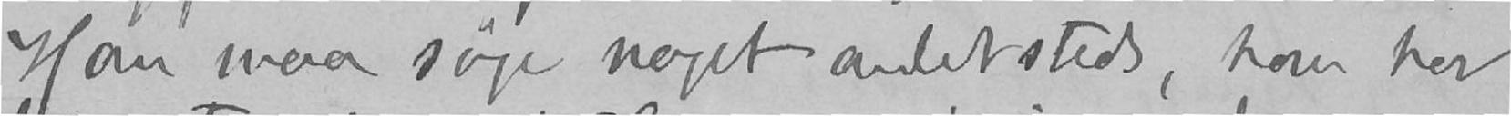Han maa søge noget andetsteds, han har
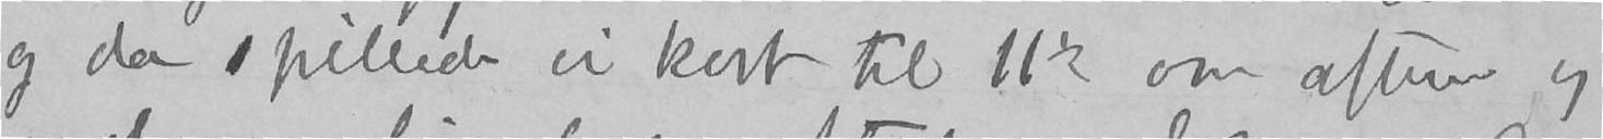og da spillede vi kort til 11½ om aftenen og
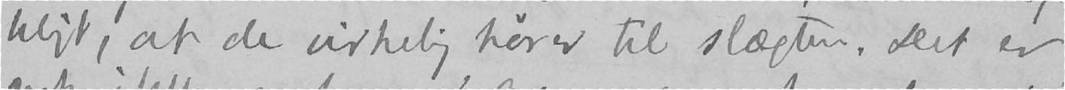teligt, at de virkelig hører til slægten. Det er
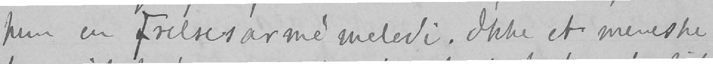hun en Frelsesarmé melodi. Ikke et menneske
